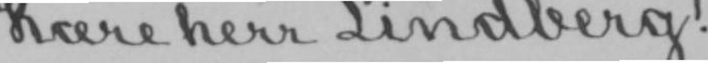Kære herr Lindberg!
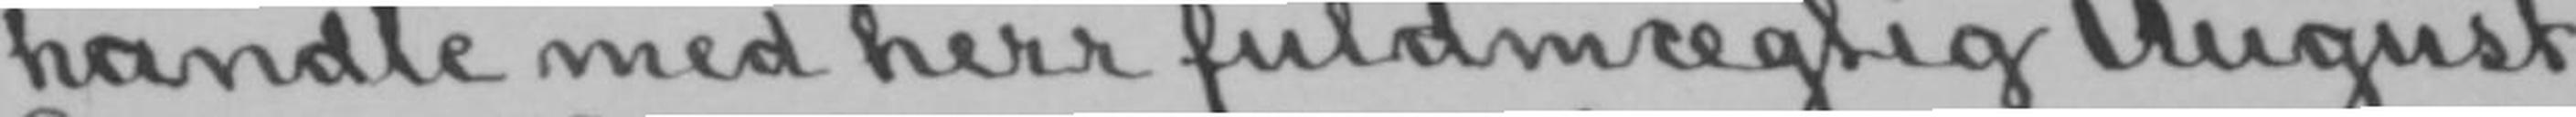handle med herr fuldmægtig August
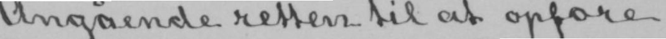Angående retten til at opføre
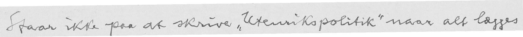Staar ikke paa at skrive "Utenrikspolitik" naar alt lægges
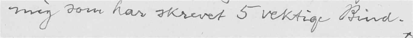mig som har skrevet 5 vektige Bind.
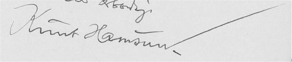Knut Hamsun.
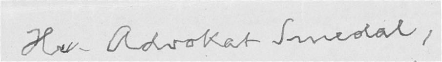Hr. Advokat Smedal,
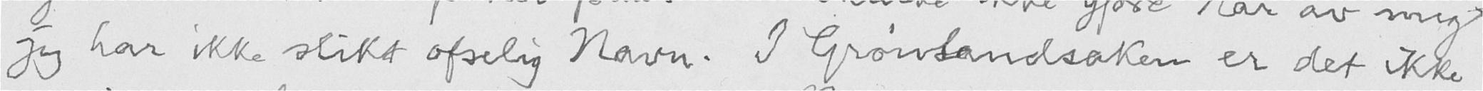jeg har ikke slikt ofselig Navn. I Grønlandsaken er det ikke
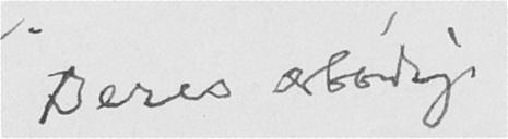Deres ærbødige
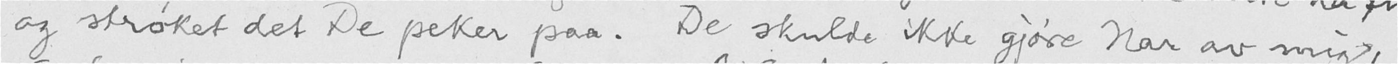og strøket det De peker paa. De skulde ikke gjøre Nar av mig,
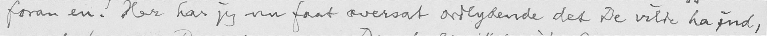foran en! Her har jeg nu faat oversat ordlydende det De vilde ha ind,
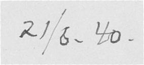21/6. 40.
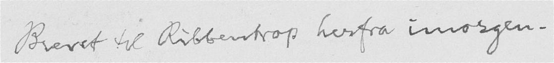Brevet til Ribbentrop herfra imorgen.
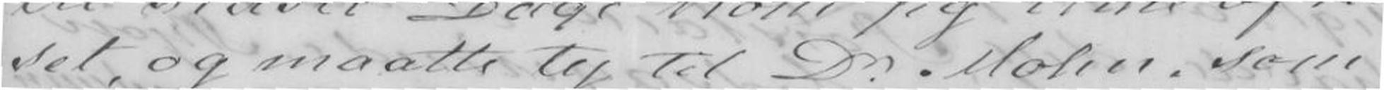set, og maatte ty til Dr Mohn, som
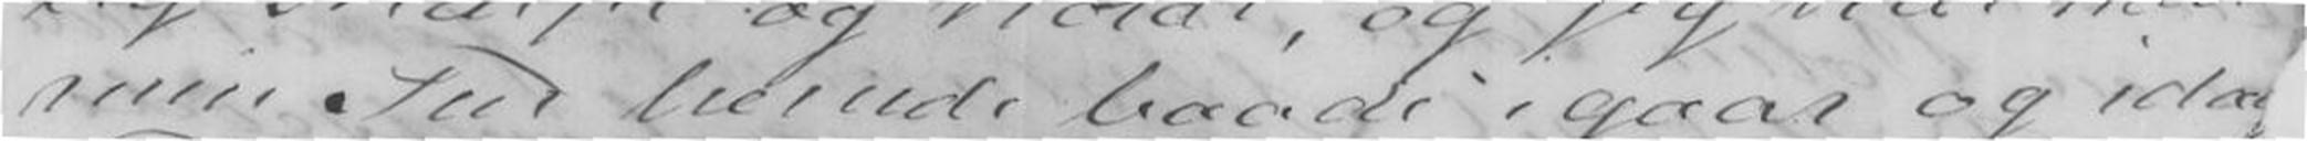min Tur herude baade igaar og idag
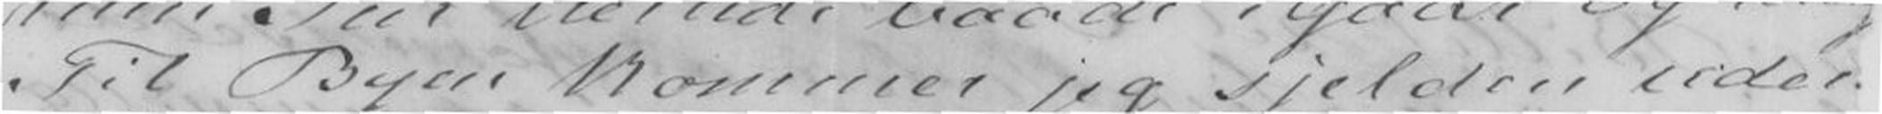Til Byen kommer jeg sjelden uden
